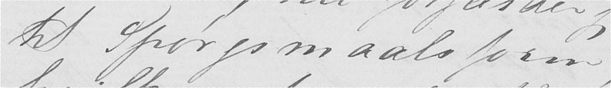til Spørgsmaalsform,
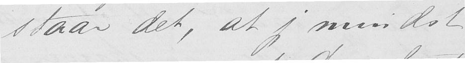staar det, at jeg mindst
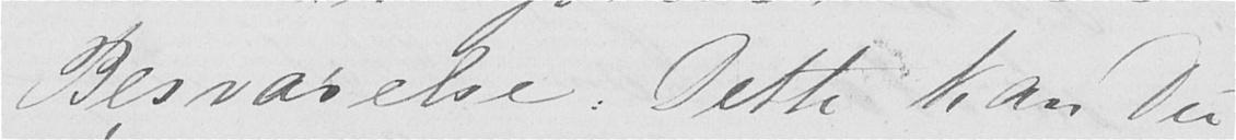Besvarelse. Dette kan Du
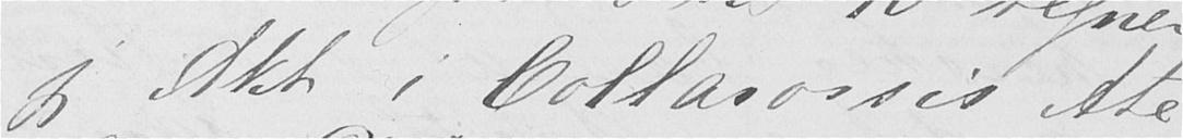jeg Akt i Collasossis Ate-
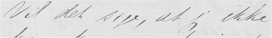Vil det sige, at jeg ikke
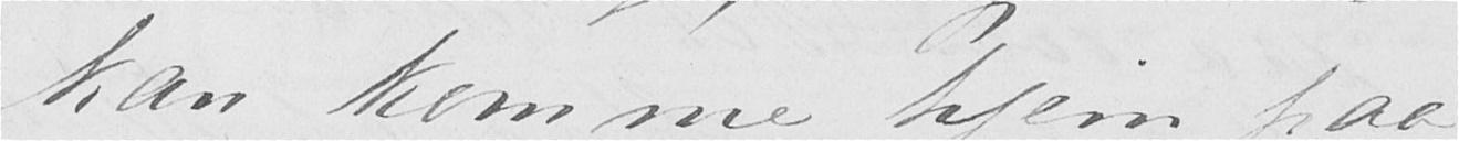kan komme hjem paa
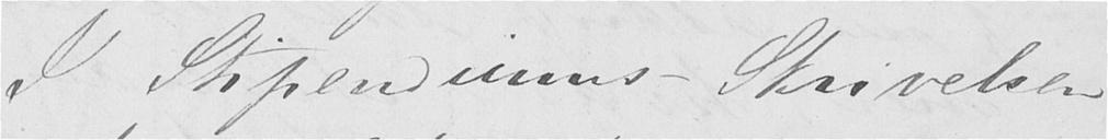I Stipendiums-Skrivelsen
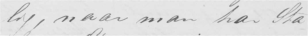lig, naar man har Sta-
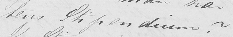tens Stipendium?
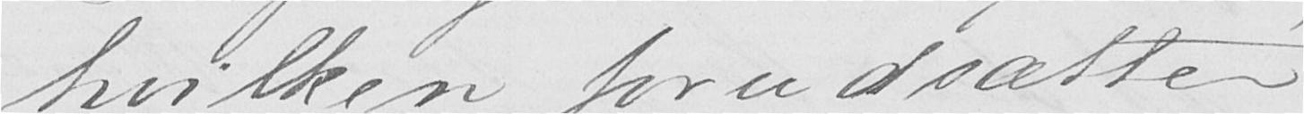hvilken forudsætter
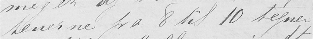tenerne fra 8 til 10 tegner,
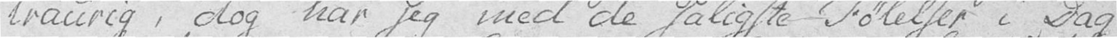traurig, dog har jeg med de saligste Følelser i Dag
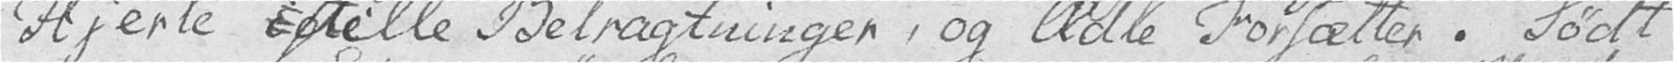Hjerte istille Betragtninger, og Ædle Forsætter. Sødt
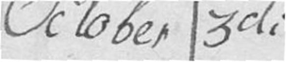October 3di
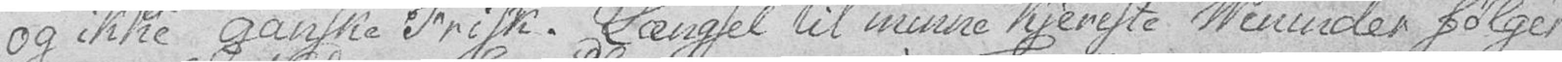og ikke ganske Frisk. Længsel til minne kjereste Veninder følger
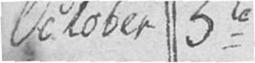October 5te
